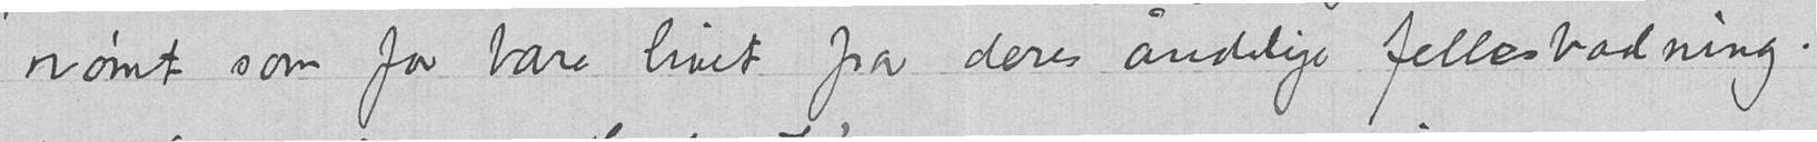rømt som for bare livet fra deres åndelige fellesbadning.
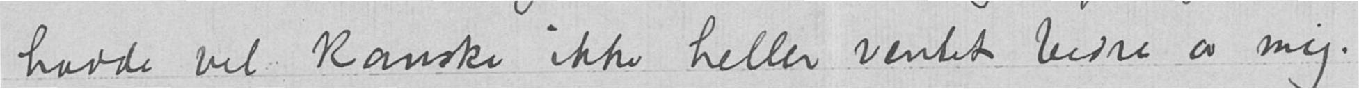hadde vel kanske ikke heller ventet bedre av mig.
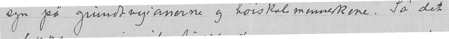syn på grundtvigianerne og høiskolmenneskene. Så det
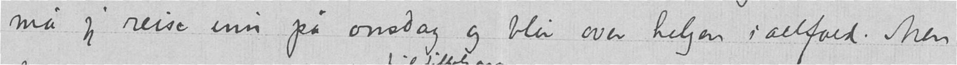må jeg reise inn på onsdag og blir over helgen iallfald. Men
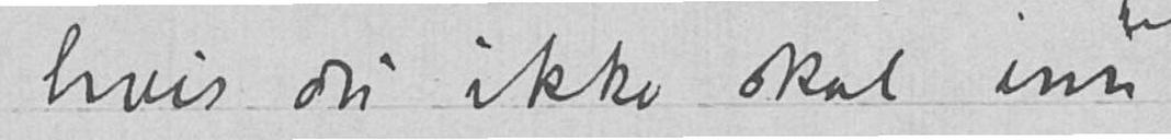hvis du ikke skal inn
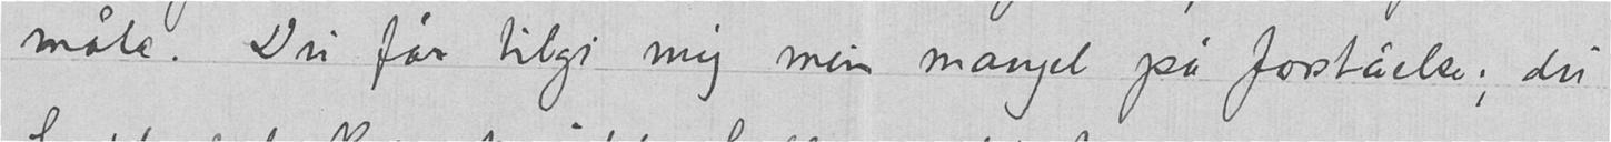måte. Du får tilgi mig min mangel pa forståelse; du
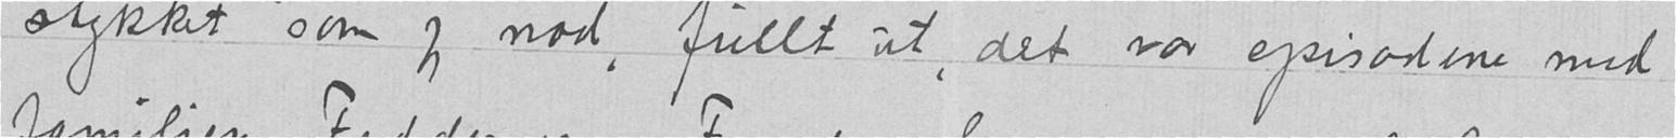stykket som jeg nød, fullt ut, det var episodene med
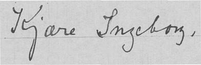Kjære Ingeborg,
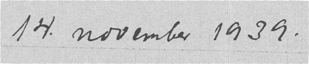14. november 1939.
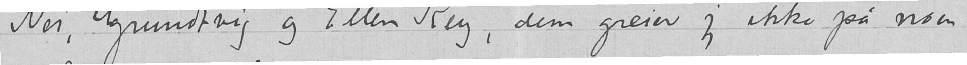Nei, Grundtvig og Ellen Key, dem greier jeg ikke på noen
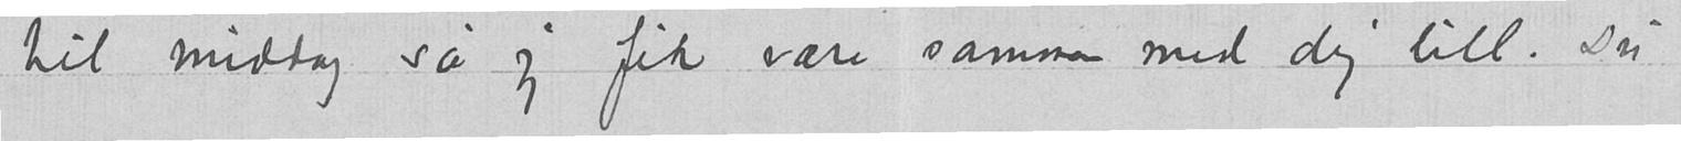til middag så jeg fik være sammen med dig litt. Du
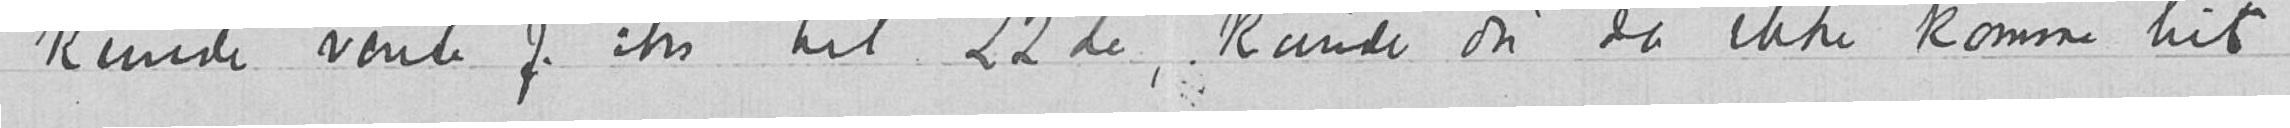kunde vente f.eks til 22de, kunde du da ikke komme hit
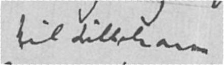til Lillehammer
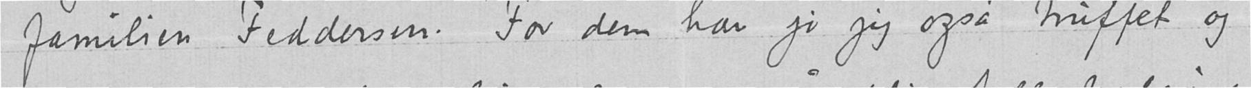familien Feddersen. For dem har jo jeg også truffet og
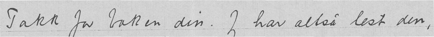Takk for boken din. Jeg har altså lest den,
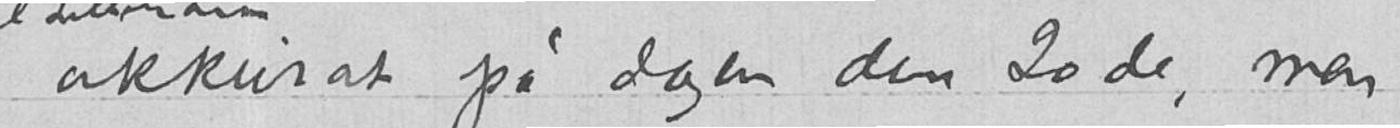akkurat på dagen den 20de, men
